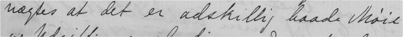nægtes at det er adskillig baade møie
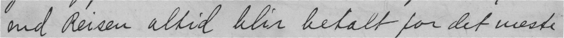end Reisen altid blir betalt for det meste
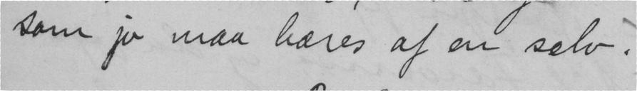som jo maa bæres af en selv.
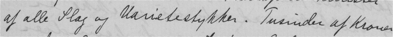af alle Slag og Varietestykker. Tusinder af Kroner
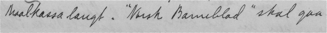Maalkassa langt. "Norsk Barneblad" skal gaa
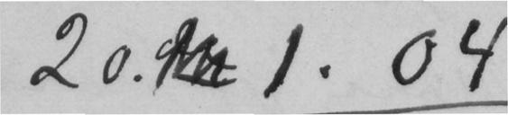20.  1. 04
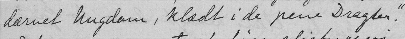dærvet Ungdom, klædt i de pene Dragter."
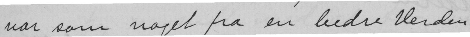var som noget fra en bedre verden
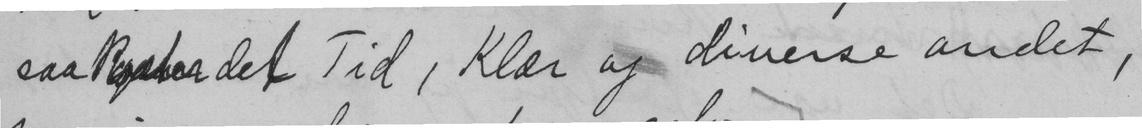saa  det Tid, Klær og diverse andet,
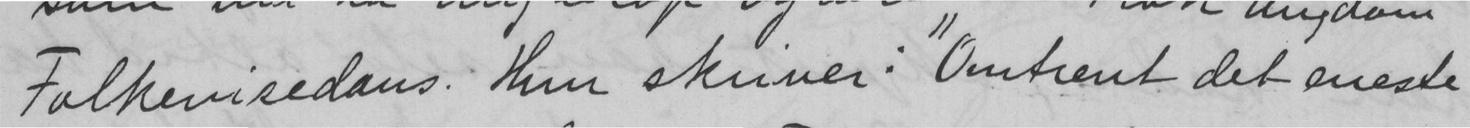Folkevisedans. Hun skriver: "Omtrent det eneste
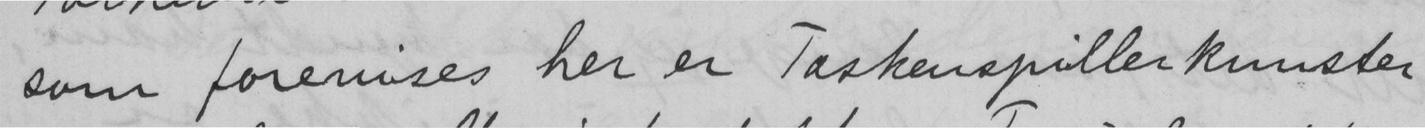som forevises her er Taskenspillerkunster
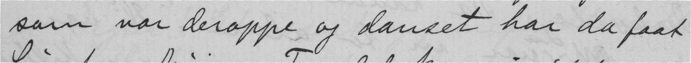som var deroppe og danset har da faat
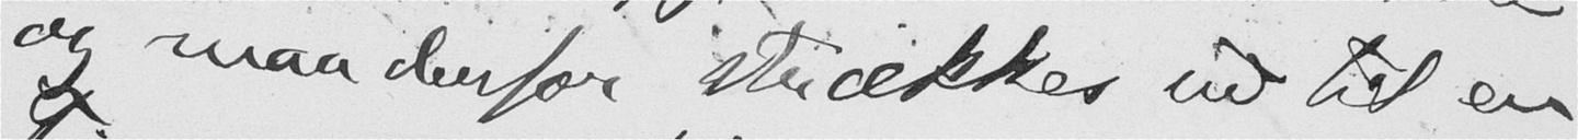og maa derfor strækkes ud til en
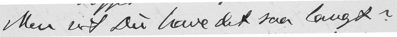Men vil Du have det saa langt?
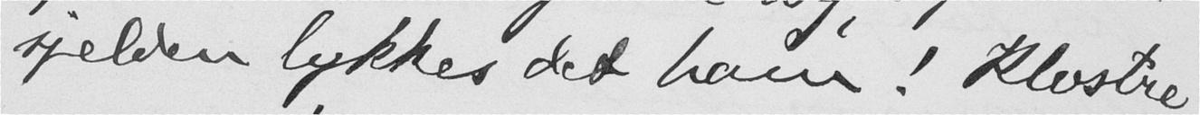sjelden lykkes det ham! Klostre
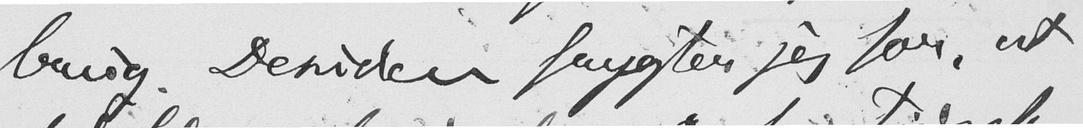brug. Desuden frygter jeg for, at
